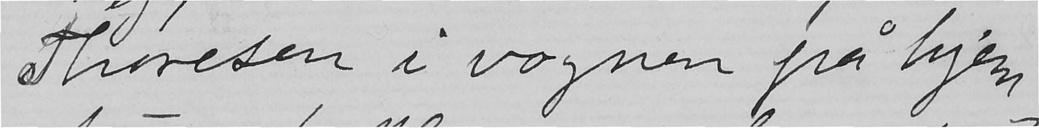Thoresen i vognen på hjem-
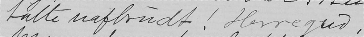talte uafbrudt! Herregud,
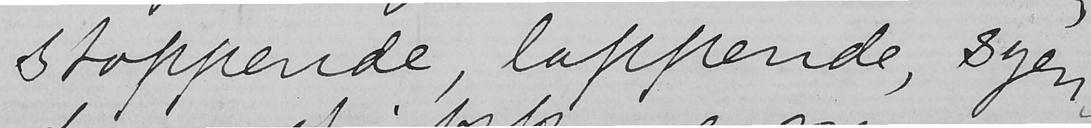stoppende, lappende syen-
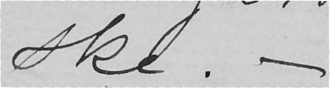ske. -
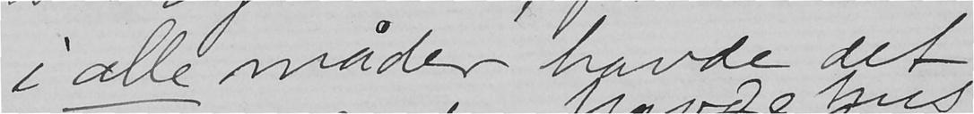i alle måder havde det
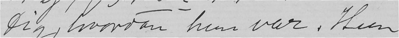Dig, hvordan hun var. Hun
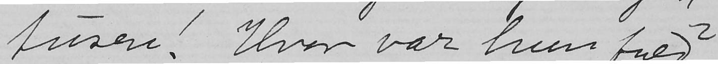turen! Hvor var hun fuld
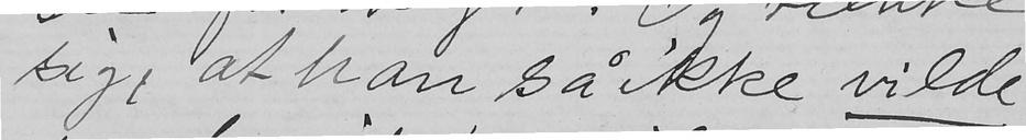sig, at han så ikke vilde
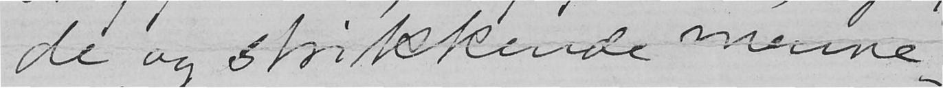de og strikkende menne-
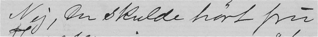Nej, Du skulde hørt fru
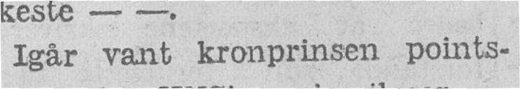Igår vant kronprinsen points-
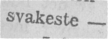svakeste - -.
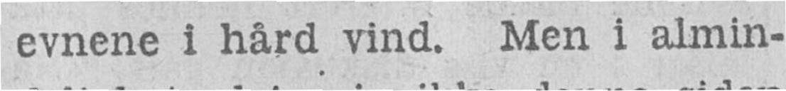evnene i hård vind. Men i almin-
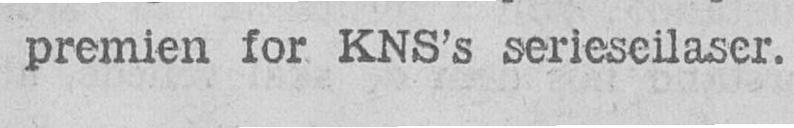premien for KNS's serieseilaser.
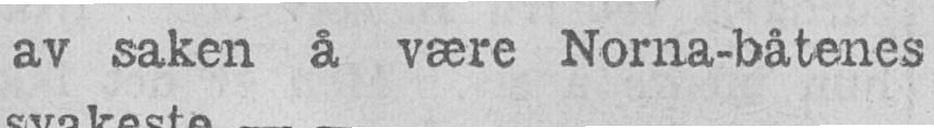av saken å være Norna-båtenes
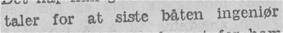taler for at siste båten ingeniør
